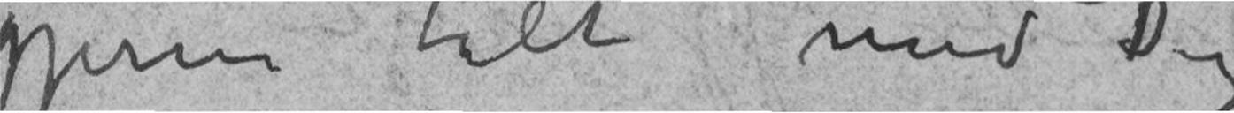gjerne talt med Dig
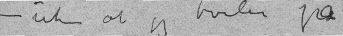– uten at jeg tviler på
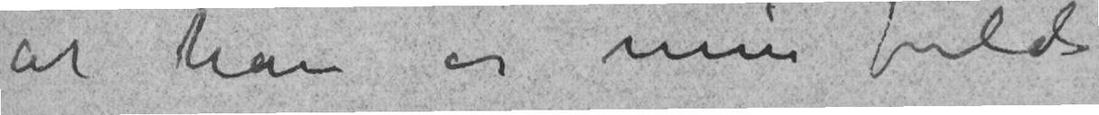at han er min fulde
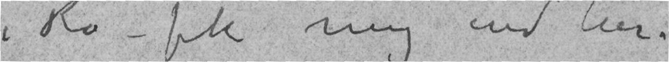i Ro – fik mig ind her.
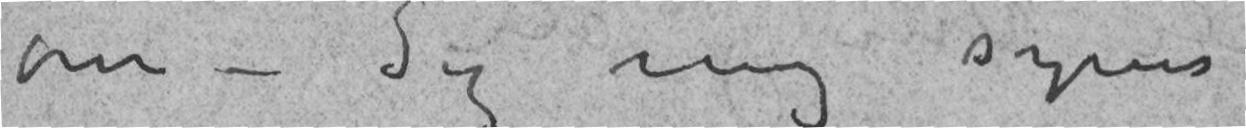om – Sig mig synes
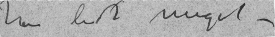han lidt meget –
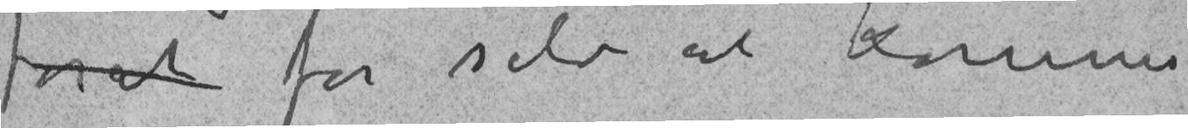forat for selv at komme
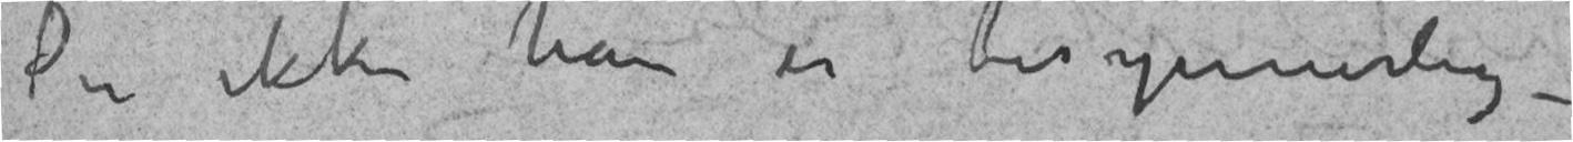Du ikke han er besynderlig –
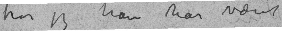tror jeg han har været
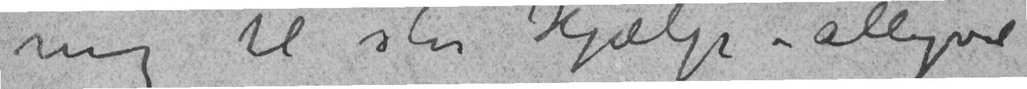mig til stor Hjælp – alligevel
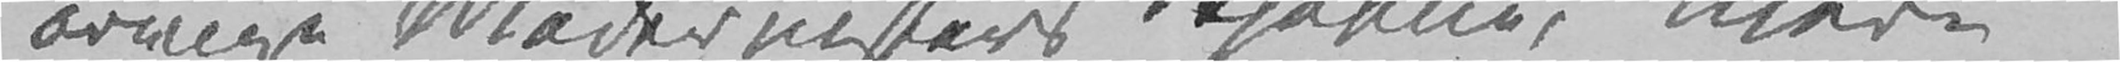øvrige Modernisters Skjebne, mere
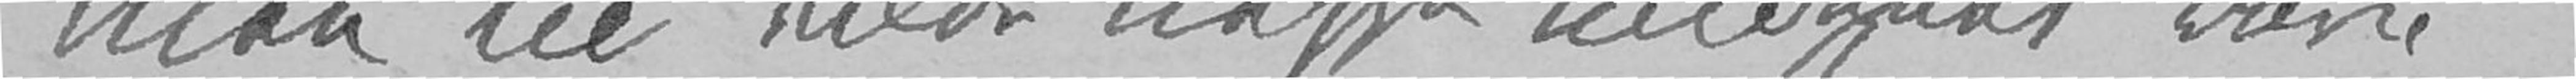men Lie vilde neppe undgået vore
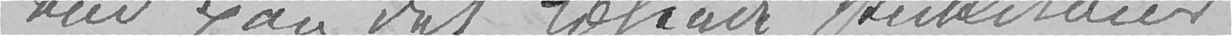end paa det læsende Publikums
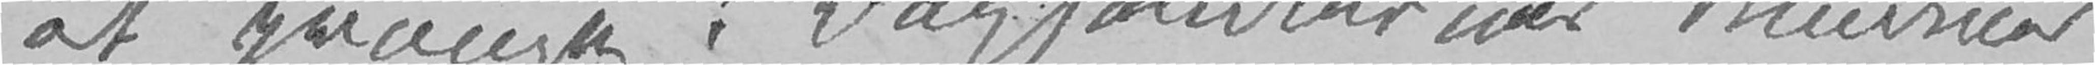at prange i Boghandlernes Vinduer
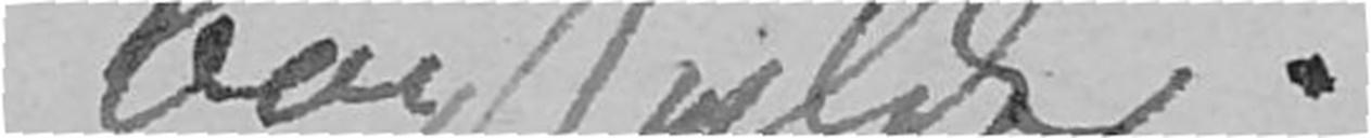Boghylder.»
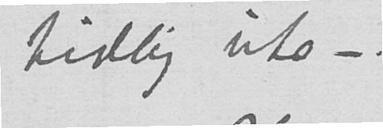tidlig ute –.
